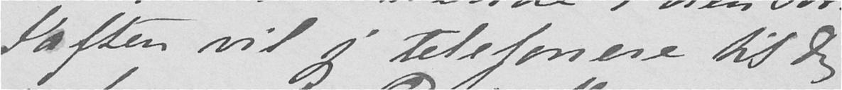Iaften vil jeg telefonere til Dig
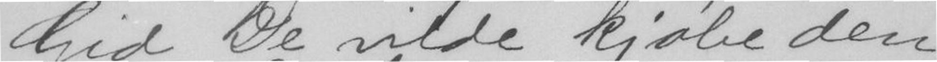Gid De vilde kjøbe den
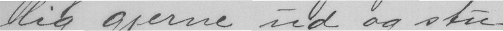lig gjerne ud og stu-
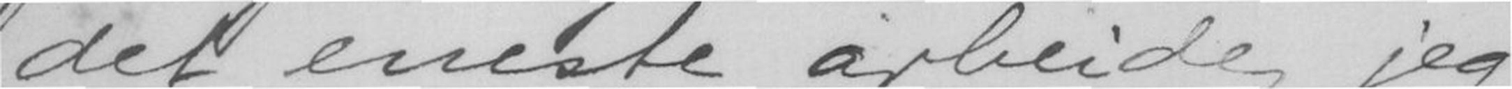det eneste arbeide jeg
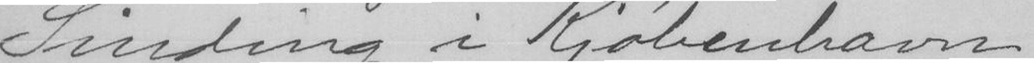Sinding i Kjøbenhavn
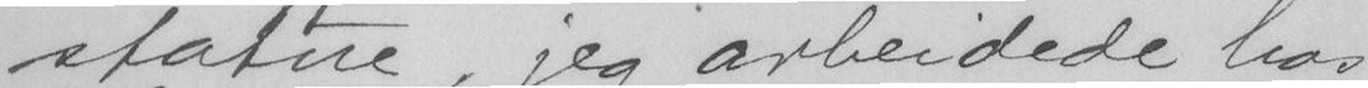statue, jeg arbeidede hos
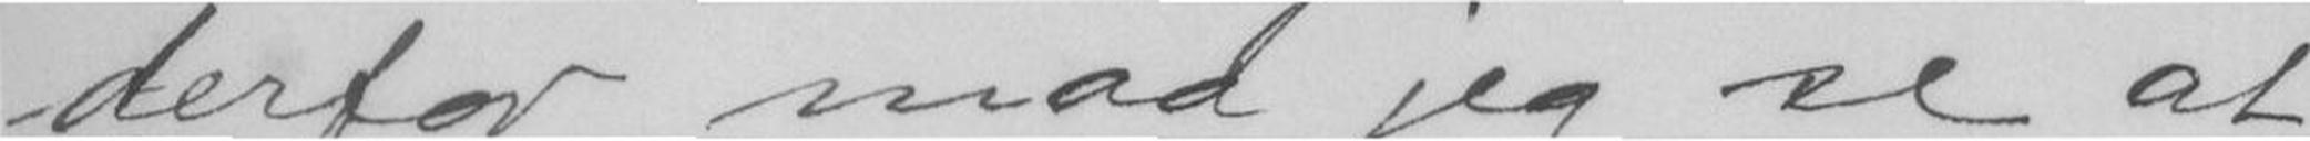derfor maa jeg se at
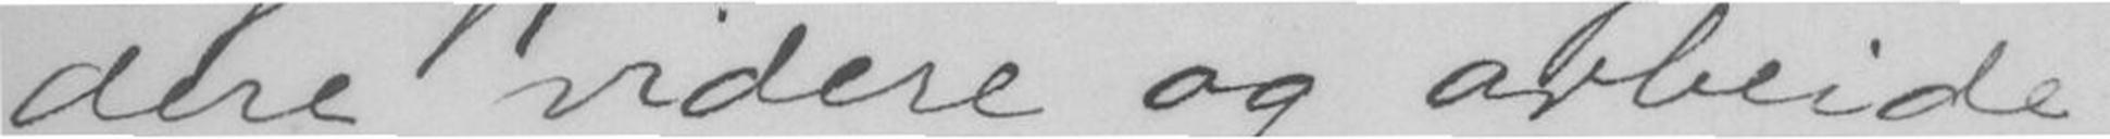dere videre og arbeide
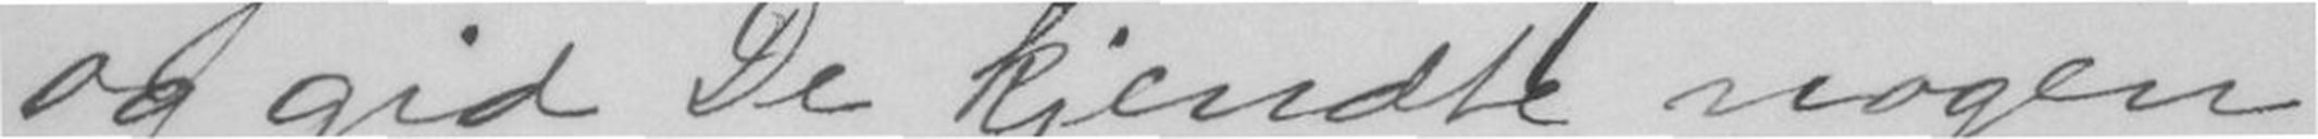og gid De kjendte nogen
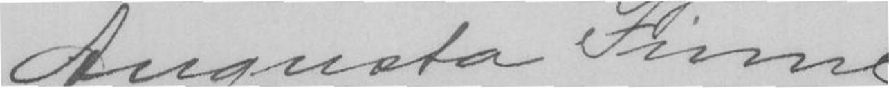Augusta Finne
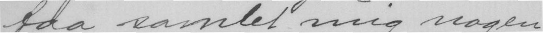faa samlet mig nogen
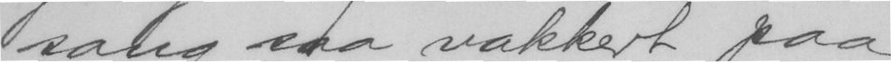sang saa vakkert paa
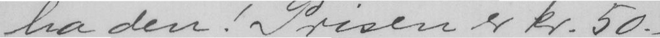ha den! Prisen er kr. 50.-
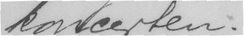koncerten.
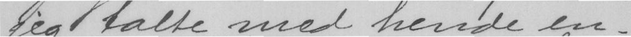jeg talte med hende en-
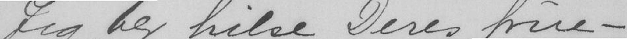Jeg ber hilse Deres frue -
